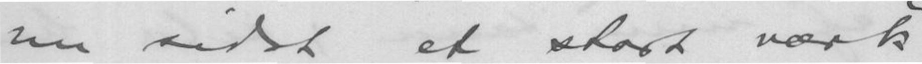nu sidst et stort værk
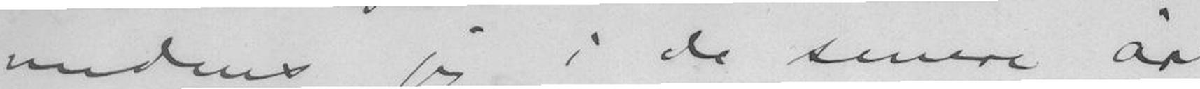medens jeg i de senere år
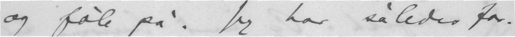og føle på. Jeg har således for-
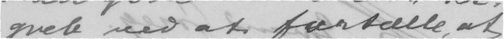greb ved at fortælle, at
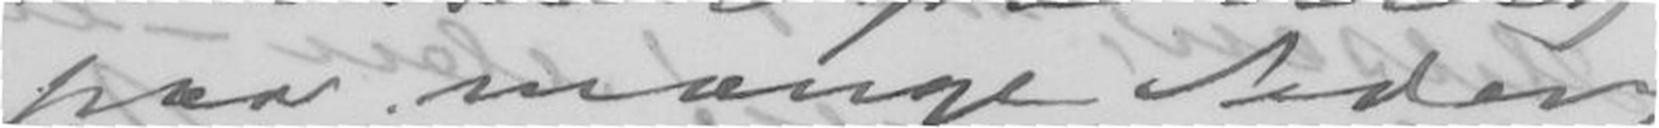paa mange Sider,
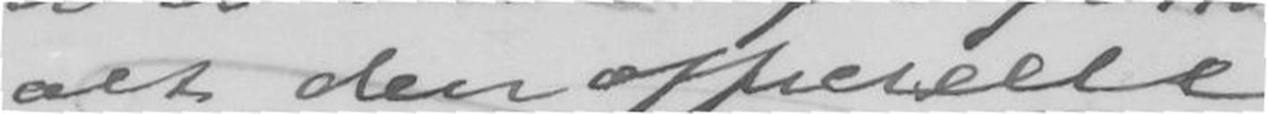- alt den offecielle
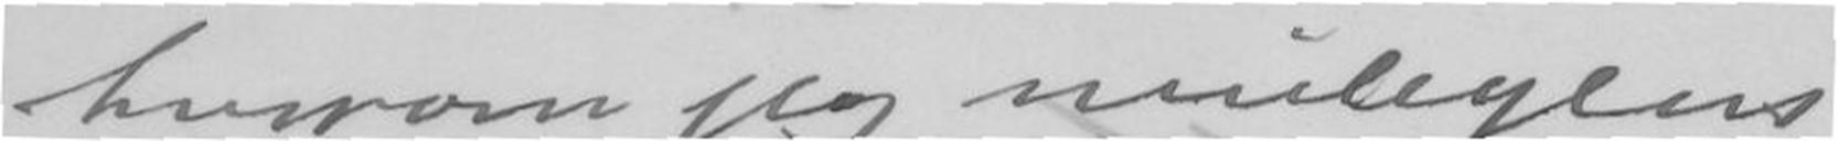hvorom jeg muligens
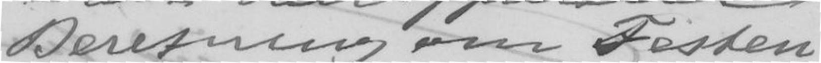Beretning om Festen
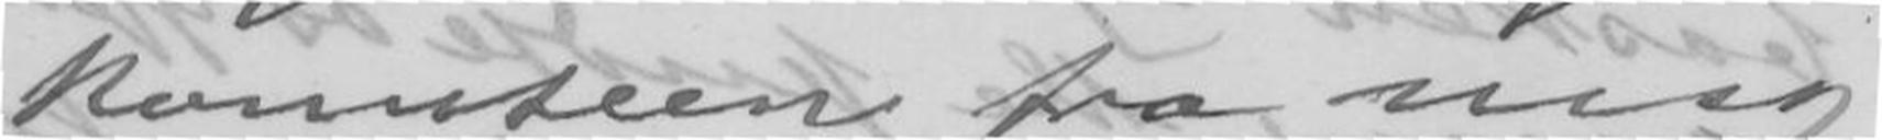komiteen fra mig
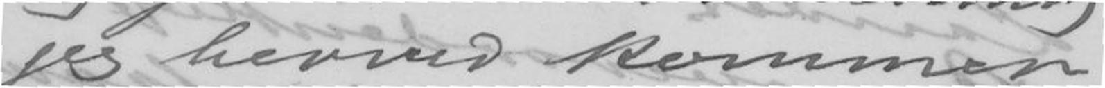jeg herved kommer
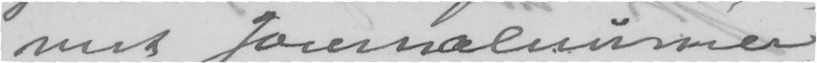mit Journalnumer
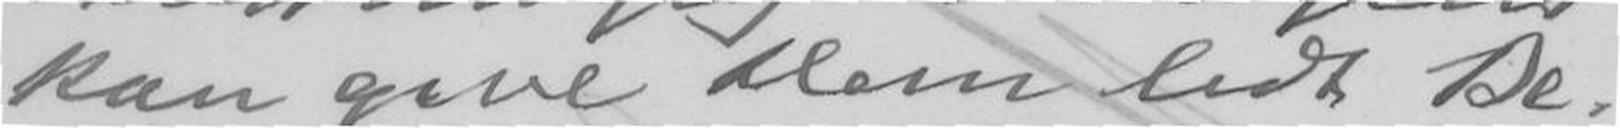kan give Dem lidt Be-
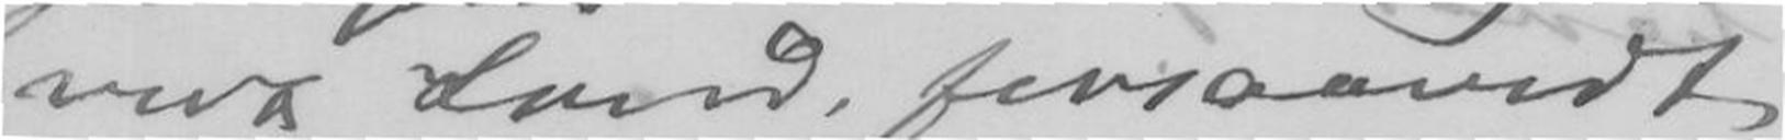vort Land forsaavidt
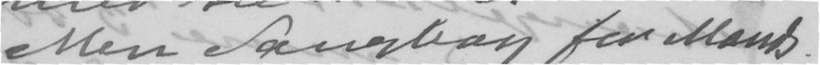Men Sangbog for Mands-
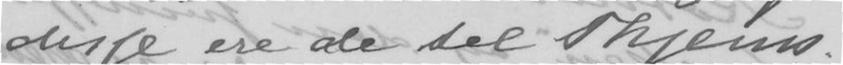disse ere de til Thjems.
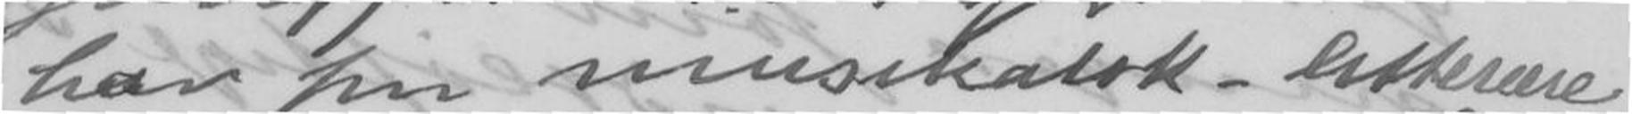har sin musikalsk - litterære
